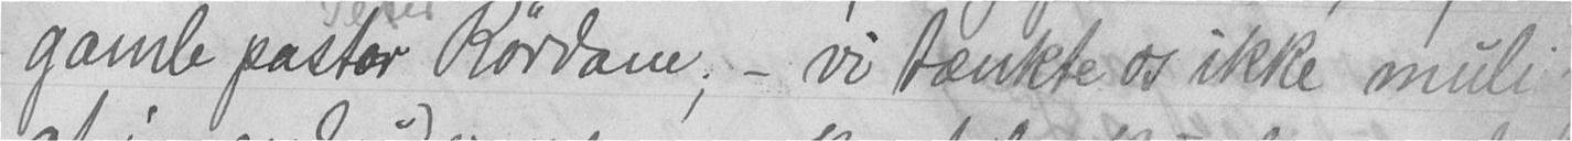gamle pastor Peter Rørdam; - vi tænkte os ikke muli
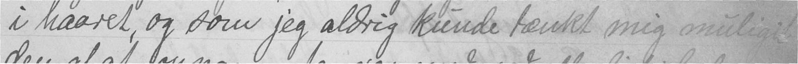i haaret, og som jeg aldrig kunde tænkt mig mulighe-
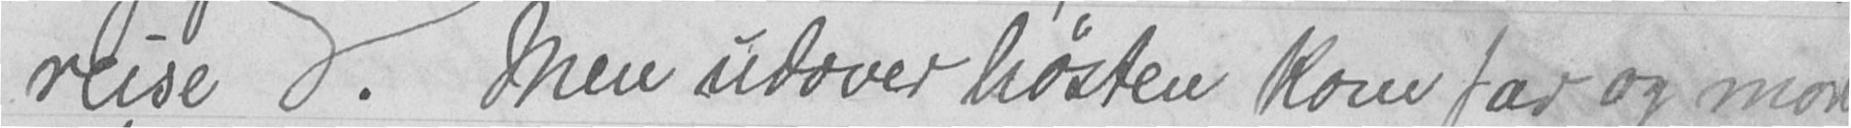reise. Men udover høsten kom far og mor
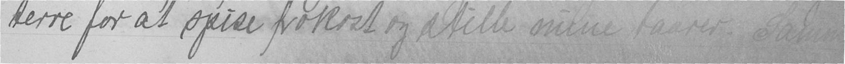terre for at spise frokost og stille mine taarer. Samme
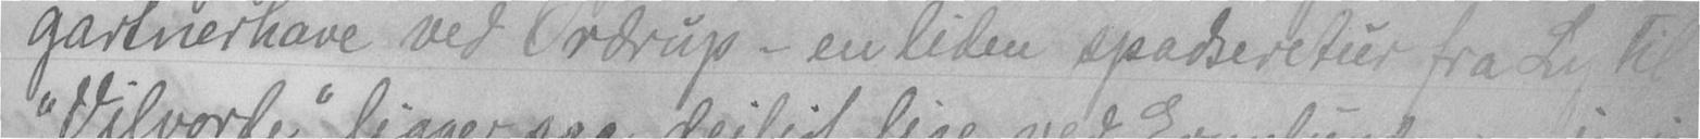gartnerhave ved Ordrup - en liden spadseretur fra Ly til
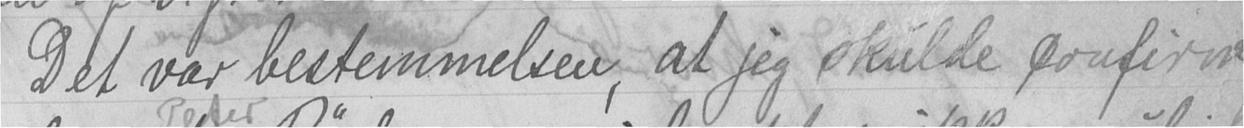Det var bestemmelsen, at jeg skulde confirm
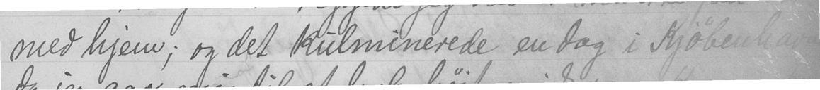med hjem; og det kulminerede en dag i Kjøbenhavn
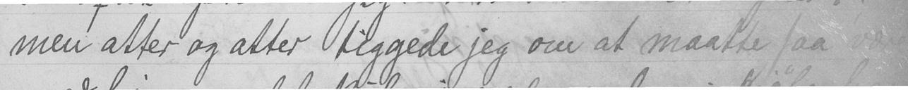men atter og after tiggede jeg om at maatte faa være
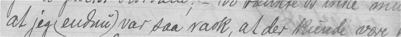at jeg endnu var saa rask, at der kunde være
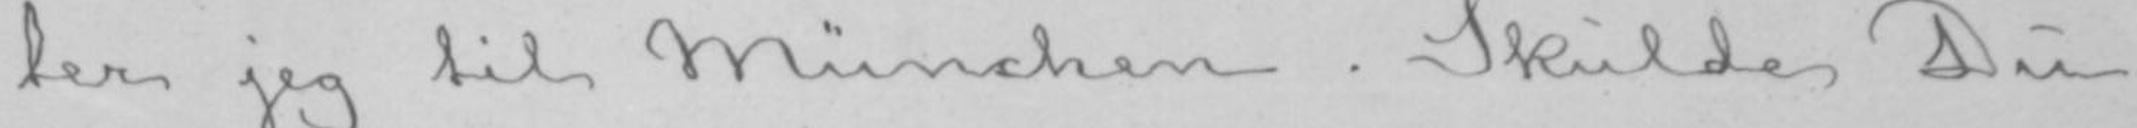ter jeg til München. Skulde Du
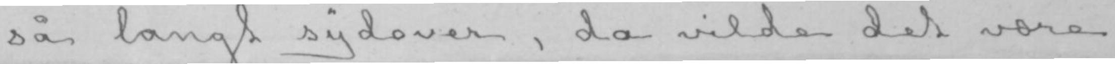så langt sydover, da vilde det være
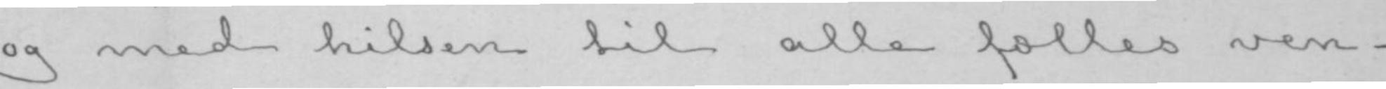og med hilsen til alle fælles ven-
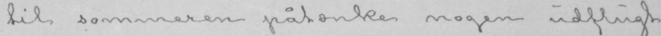til sommeren påtænke nogen udflugt
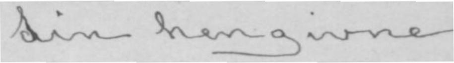din hengivne
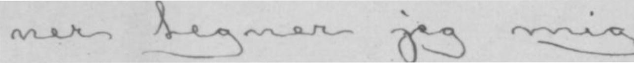ner tegner jeg mig
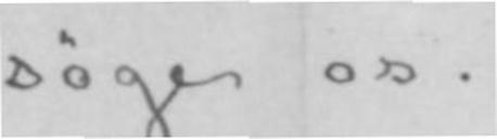søge os.
